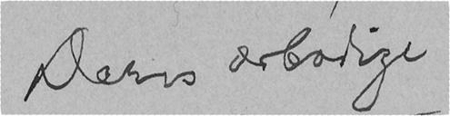Deres ærbødige
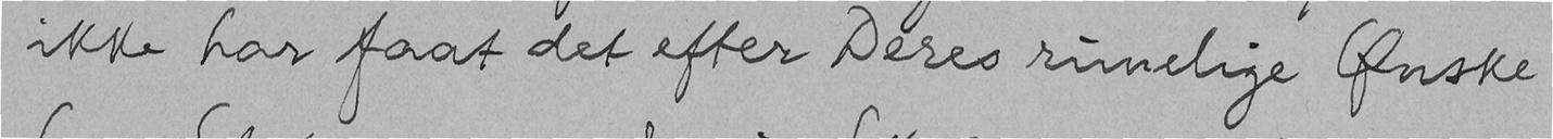ikke har faat det efter Deres rimelige Ønske
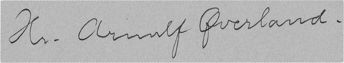Hr. Arnulf Øverland.
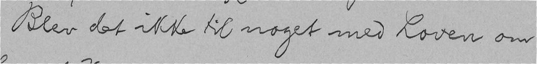Blev det ikke til noget med Loven om
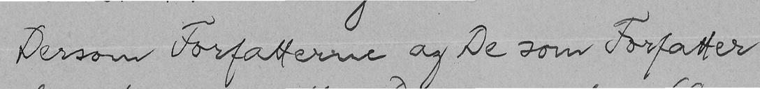Dersom Forfatterne og De som Forfatter
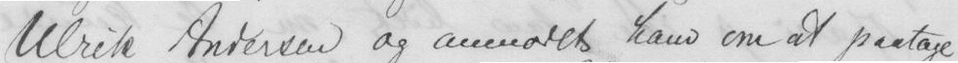Ulrik Andersen og anmodet ham om at paatage
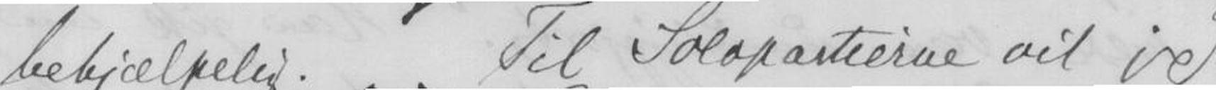behjælpelig.Til Solopartierne vil jeg
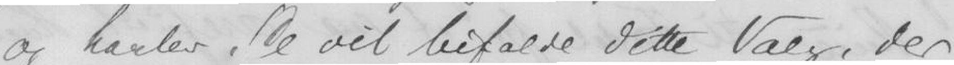og haaber, De vil vifalde dette Valg, der
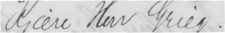Kjære Herr Grieg!
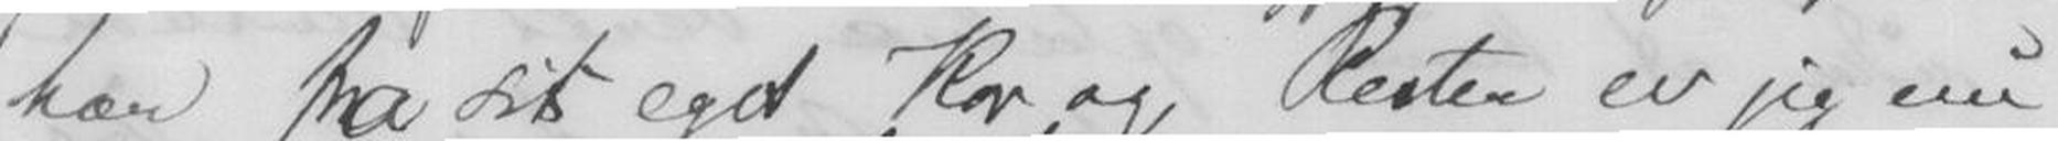har fra sit eget kor, og Resten er jeg nu
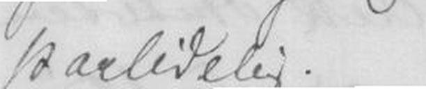paalidelig.
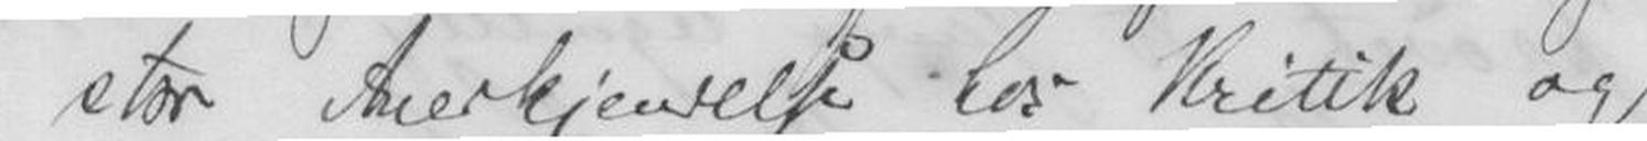stor Anerkjendelse Kritikk og
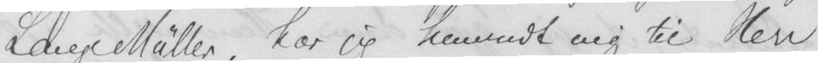Lang Müller, kor jeg henvendte mig til Herr
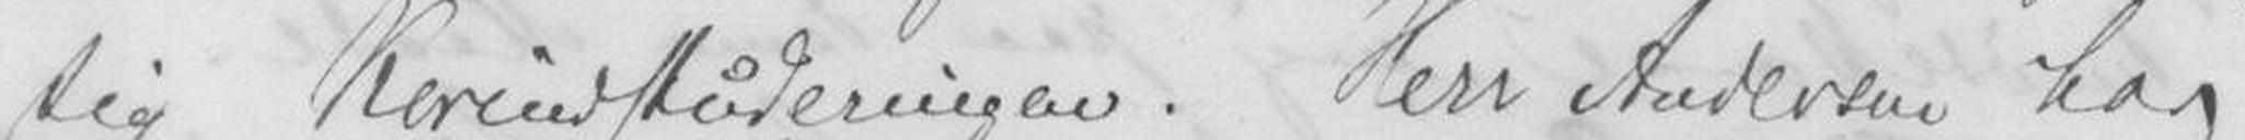sig Korindstuderingen. Herr Andersen har
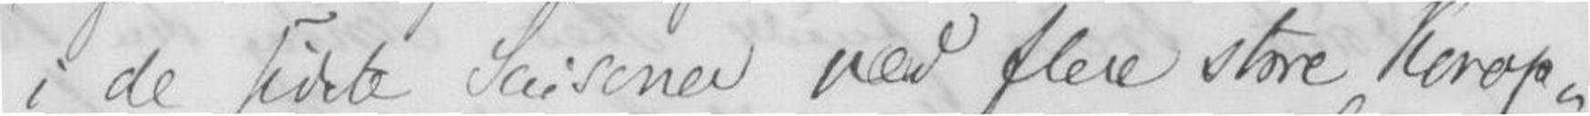i de sidste Saisoner paa flere store Korop-
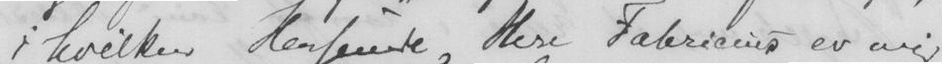i hvilken Henseende herr Fabricius er mig
